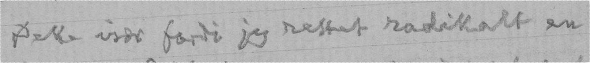Dette især fordi jeg rettet radikalt en
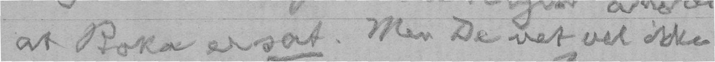at Boka er sat. Men De vet vel ikke
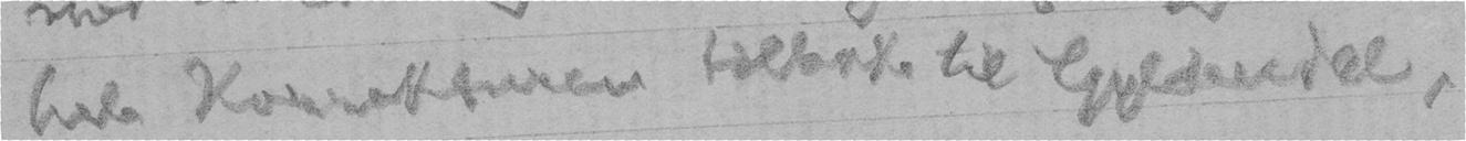hele Korrekturen tilbake til Gyldendal,
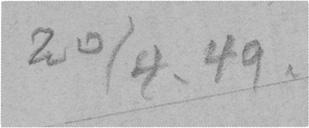20/4. 49.
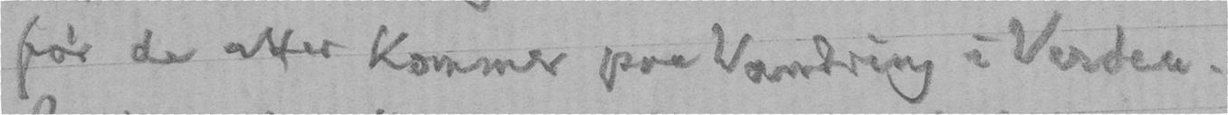før de atter kommer paa Vandring i Verden.
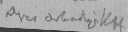Deres ærbødige KH
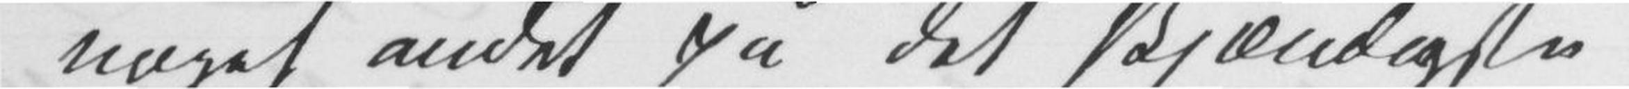noget andet på det skjændigste
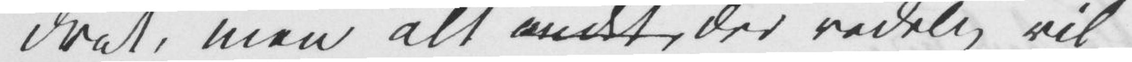dret, men alt andet der redelig vil
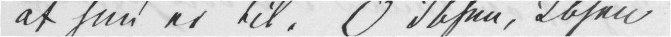at hun er til. O Ibsen, Ibsen
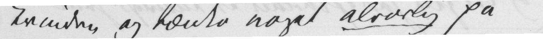Kvinden» og tænke noget alvorlig på
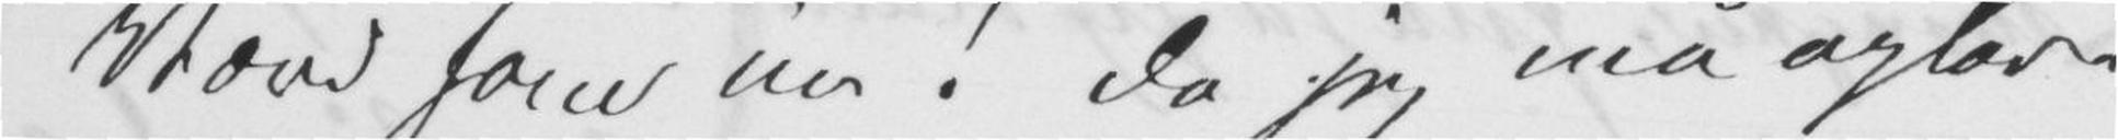Værd som nu! Da jeg må opleve
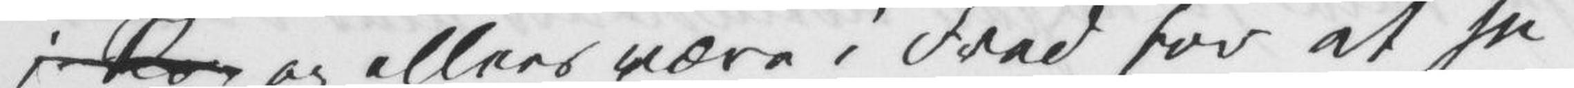i Ro og ellers være i Fred for at «se
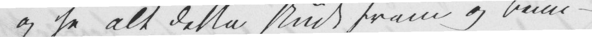og se alt dette skudt frem og beun¬
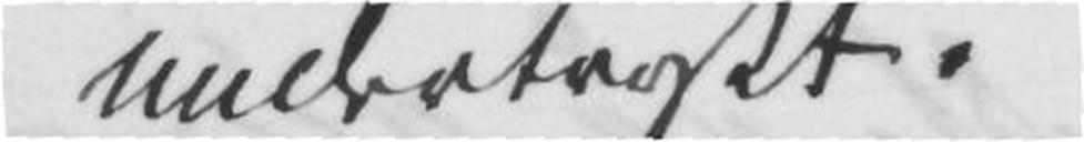undertrykt.
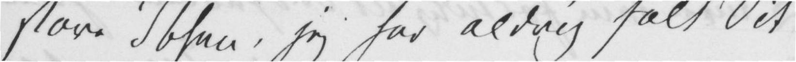store Ibsen, jeg har aldrig følt Dit
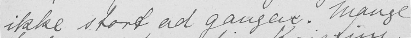ikke stort ad gangen. Mange
